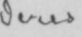Deres
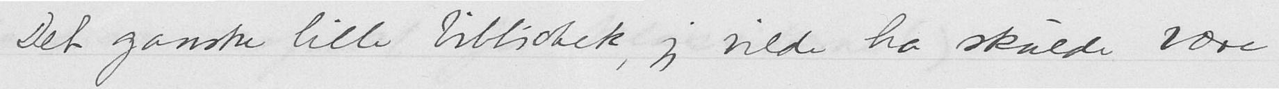Det ganske lille bibliotek, jeg vilde ha skulde være
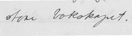store bokskapet.
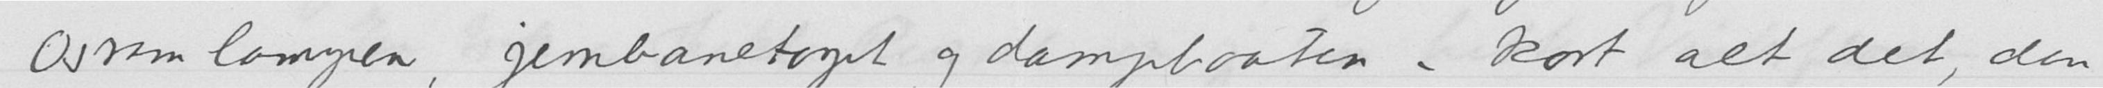Osramlampen, jembanetoget og dampbaaten – kort alt det, den
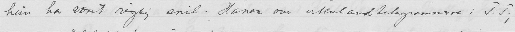hun har været rigtig snil. Hanen over utenlandstelegrammerne i T.T.,
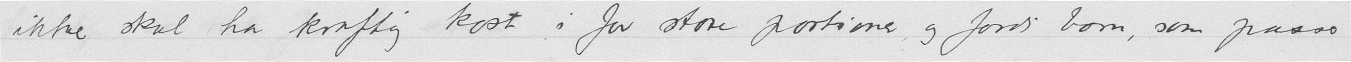ikke skal ha kraftig kost i for store portioner, og fordi barn, som passer
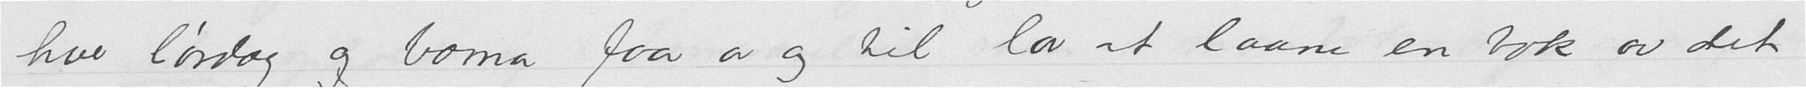hver lørdag og barna faar av og til lov at laane en bok av det
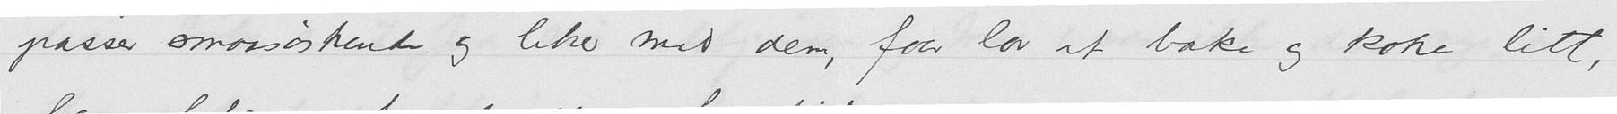passer smaasøskende og leker med dem, faar lov at bake og koke litt,
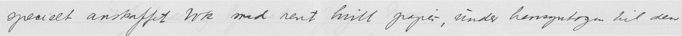specielt anskaffet bok med rent hvitt papir, under hensyntagen til den
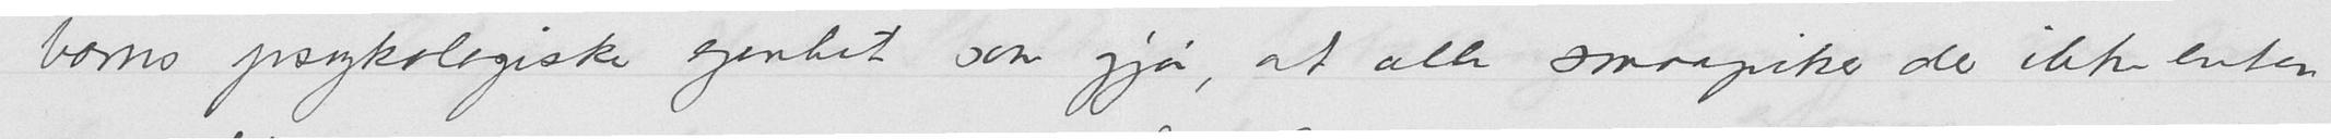barns psykologiske egenhet som gjør, at alle smaapiker der ikke enten
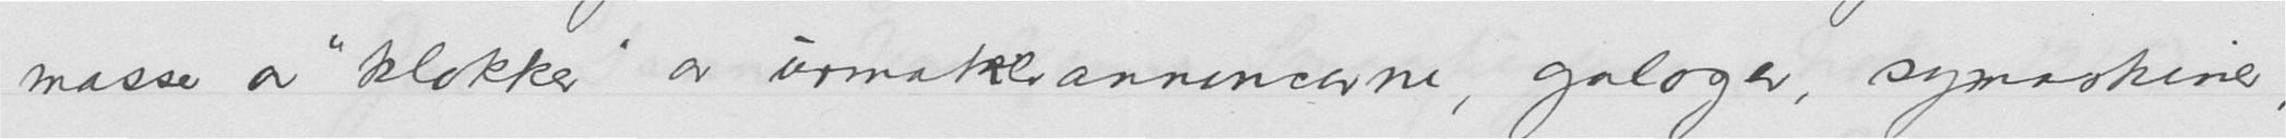masser av "klokker" av urmakerannoncerne, galoger, symaskiner,
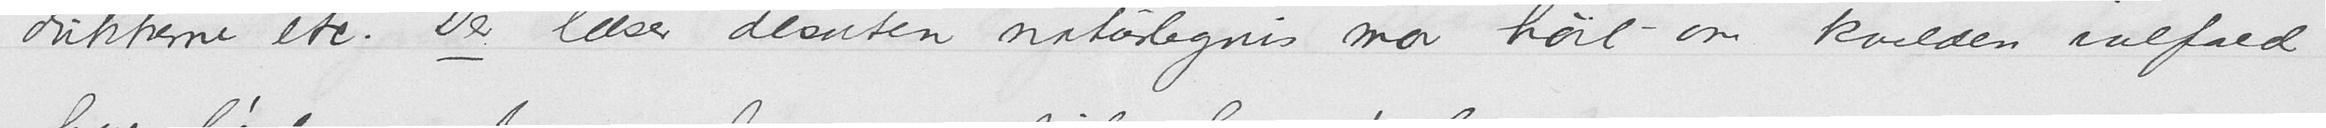dukkerne etc. Der læser desuten naturligvis mor høit om kvelden ialfald
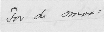For de smaa:
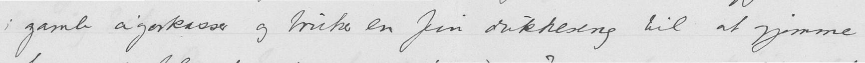i gamle cigarkasser og bruker en fin dukkeseng til at gjemme
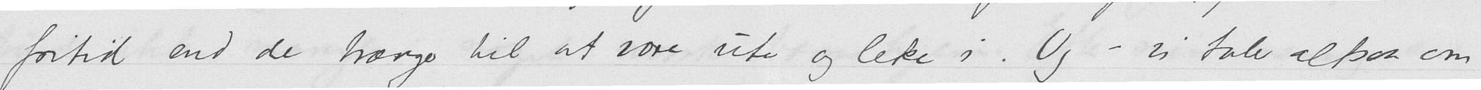fritid end de trænger til at være ute og leke i. Og – vi taler altsaa om
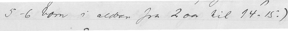5-6 barn i alderen fra 2 aar til 14-15.)
